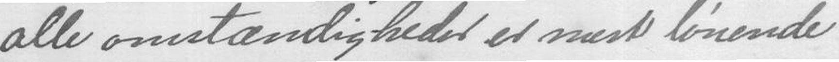alle omstændigheder er mest lønende.
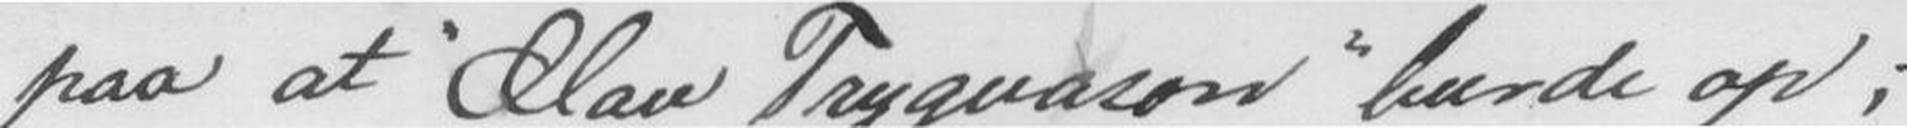paa at "Olav Trygvason" burde op;
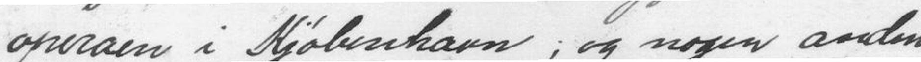operaen i Kjøbenhavn; og nogen anden
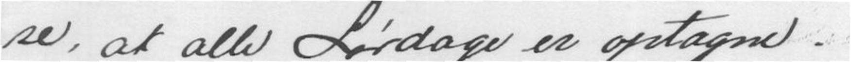se, at alle Lørdager er optagne.
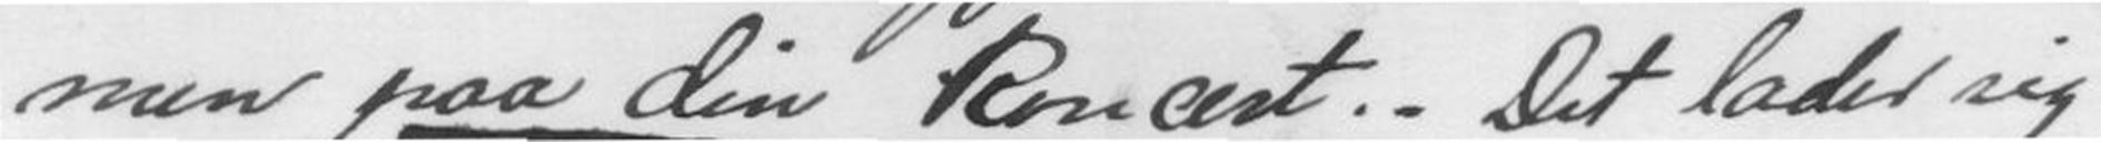men paa din koncert. - Det lader sig
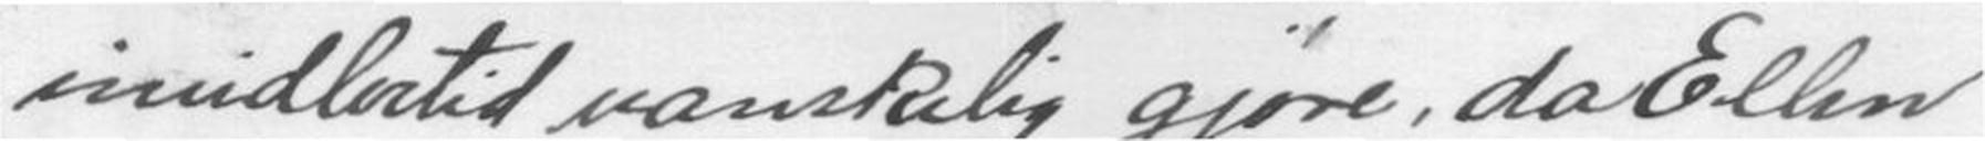imidlertid vanskelig gjøre, da Ellen
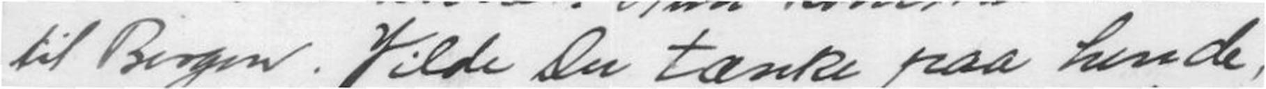til Bergen. Vilde Du tænke paa hende,
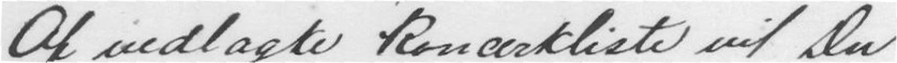Af vedlagte koncertliste vil Du
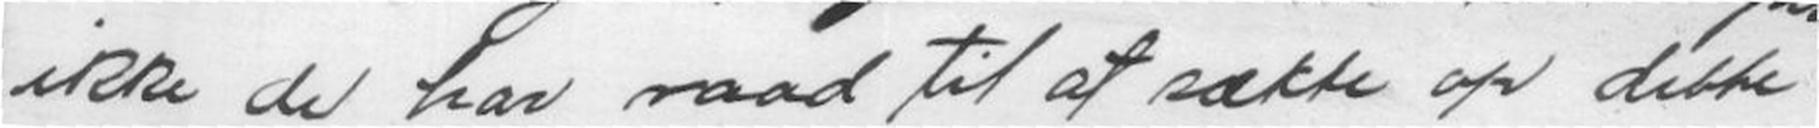ikke de har raad til at sætte op dette
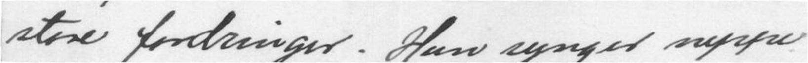store fordringer. Hun synger neppe
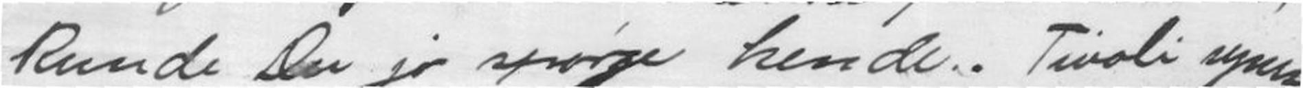kunde Du jo spørge hende. Tivoli synes
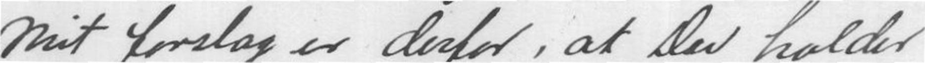Mit forslag er derfor, at Du holder
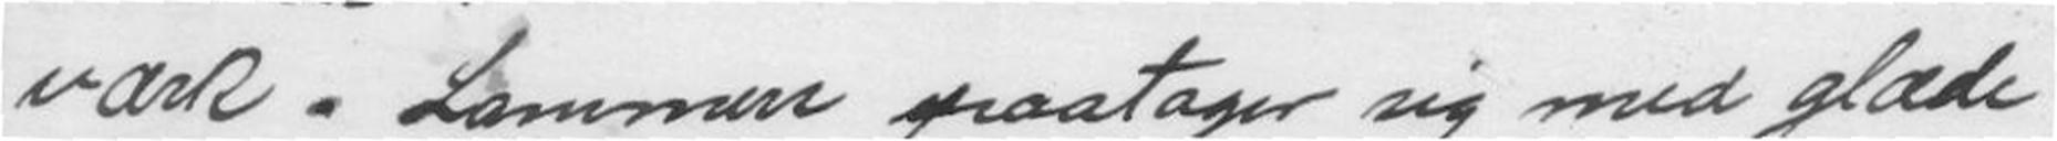værk. Lammers paatager sig med glæde
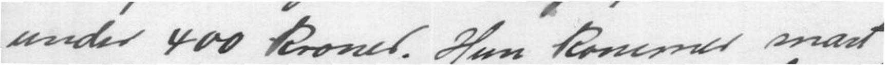under 400 kroner. Hun kommer snart
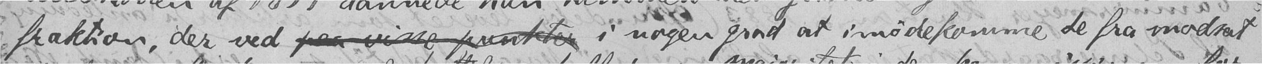fraktion, der ved  i nogen grad at imødekomme de fra modsat
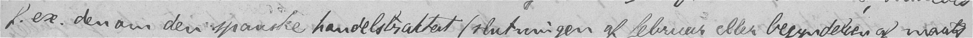f.ex. den om den spanske handelstraktat (slutningen af februar eller begyndelsen af marts
